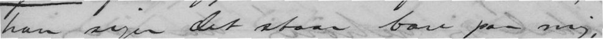han siger det staar bare paa mig,
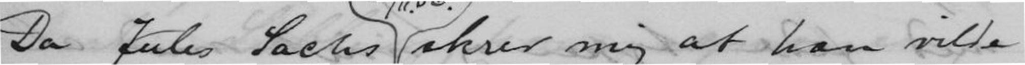Da Jules Sachs skrev mig at han vilde
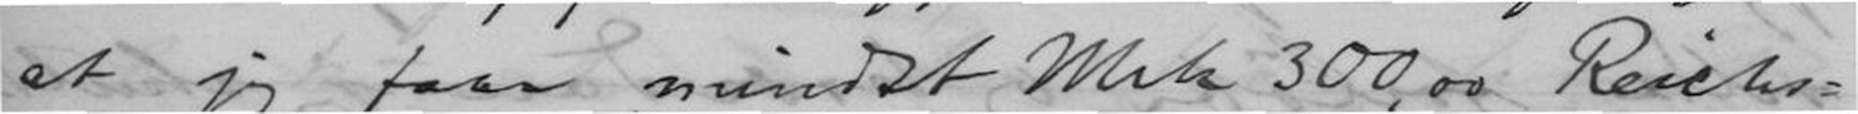at jeg faar "mindst Mrk 300.00 Reichs-
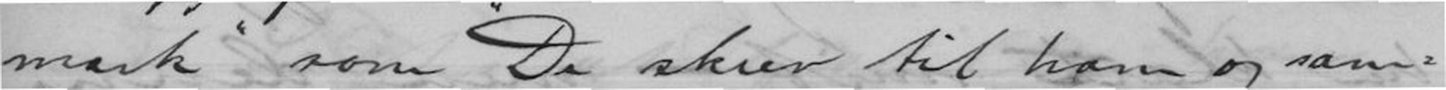mark" som De skrev til ham og sam-
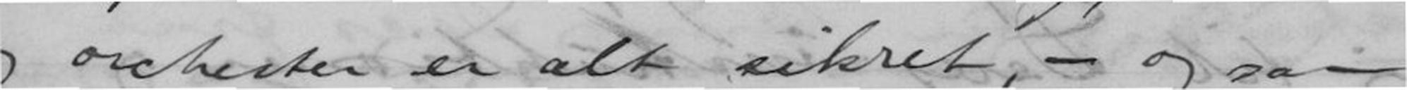og orchester er alt sikret, - og saa
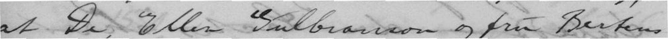at De, Ellen Gulbranson og fru Bertens
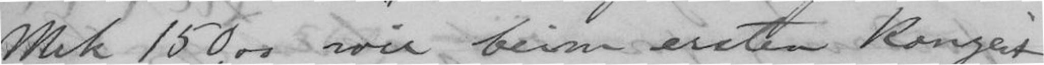Mrk 150,00 wie beim ersten Konzert"
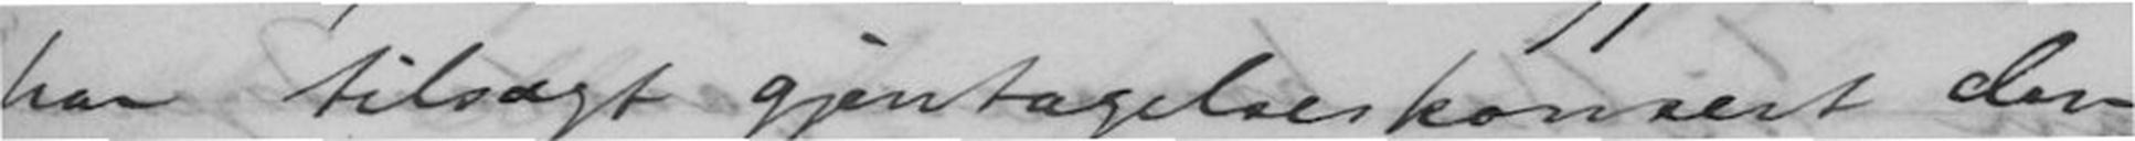har tilsagt gjentagelseskonsert den
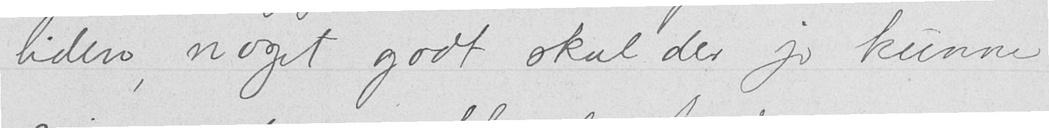liden, noget godt skal der jo kunne
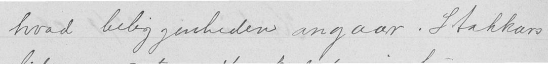hvad beliggenheden angaar. Stakkars
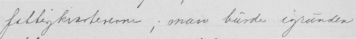fattigkvartererne; man burde igrunden
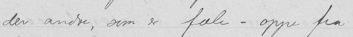er der andre, som er fæle - oppe fra
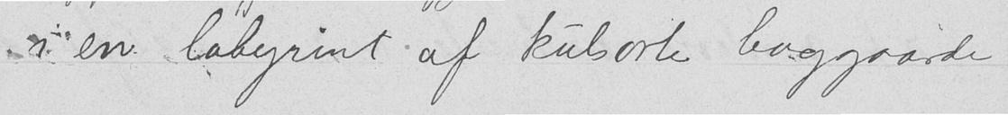i en labyrint af kulsorte baggaarde
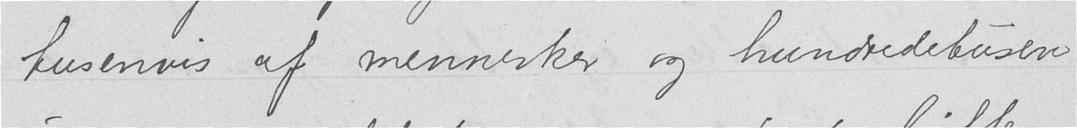tusenvis af mennesker og hundretusen
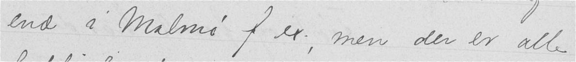end i Malmø f ex., men der er alle
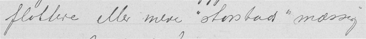flottere eller mere "storstads" mæssig
